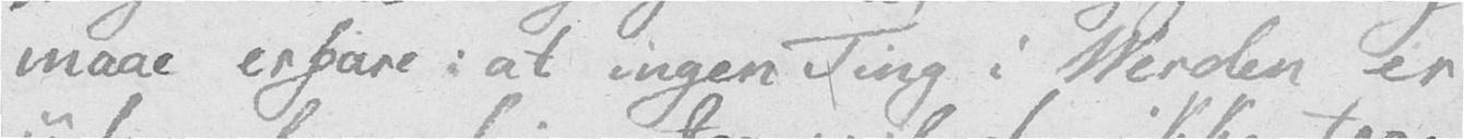maae erfare: at ingen Ting i Verden er
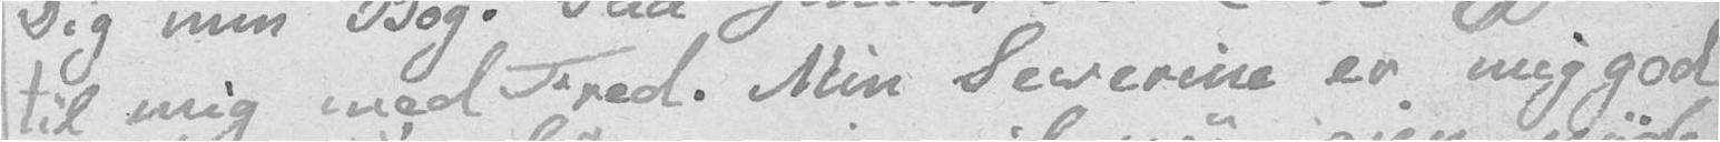til mig med Fred. Min Severine er mig god
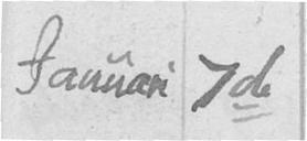Januari 7de
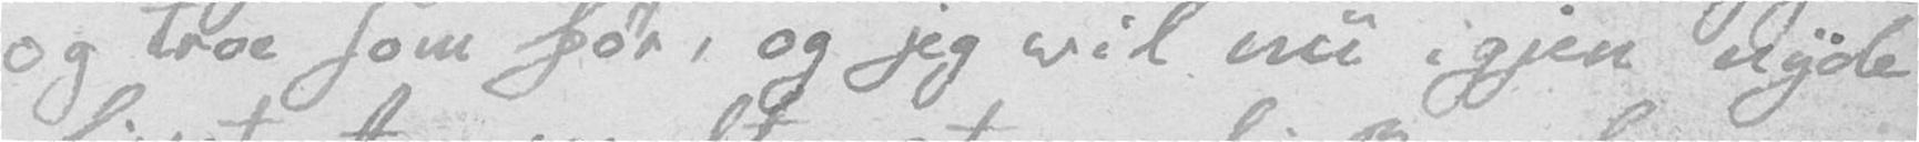og troe som før, og jeg vil nu igjen nyde
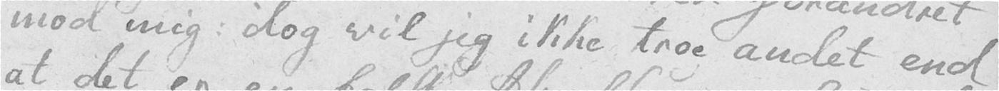mod mig: dog vil jeg ikke troe andet end
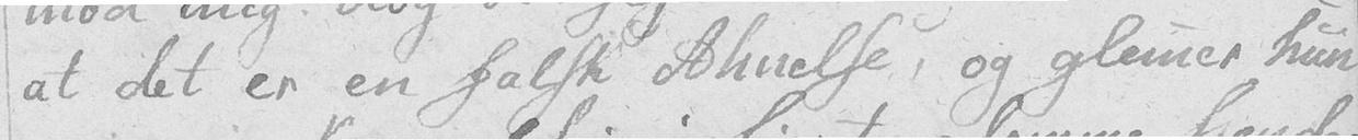at det er en falsk Ahnelse, og glemmer hun
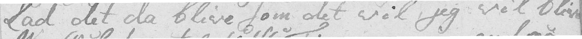Lad det da blive som det vil, jeg vil blive
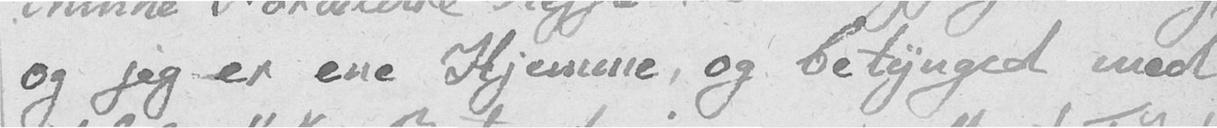og jeg er ene Hjemene, og betynged med
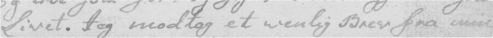Livet. Jeg modtog et venlig Brev fra min
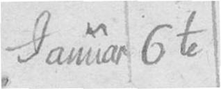Januar 6te
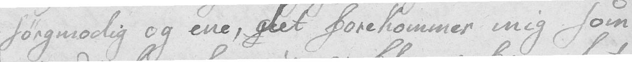sørgmodig og ene, det forekommer mig som
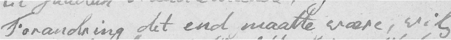Forandring det end maatte være, vil
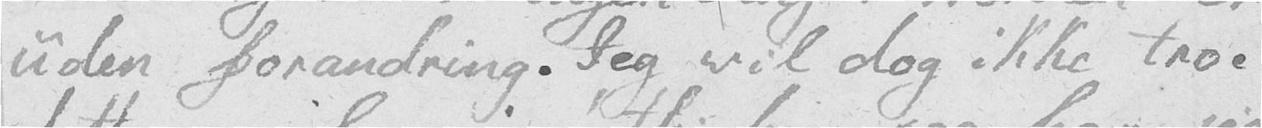uden forandring. Jeg vil dog ikke troe
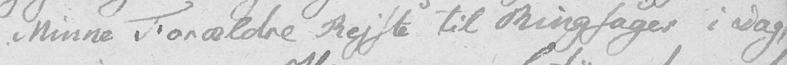Minne Forældre Rejste til Ringsager i Dag,
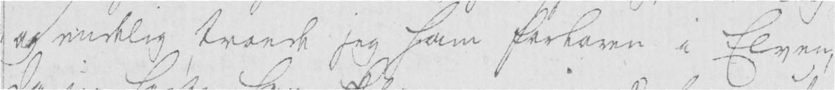og endelig troede jeg ham forloren i Elven,
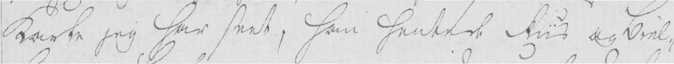Karle jeg har seet, han hentede Riis og Biel-
